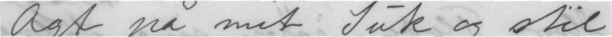Agt på mit Suk og stil
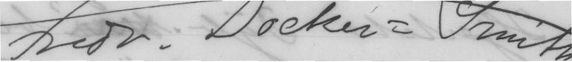Fredr. Döcker=Smith
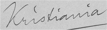Kristiania
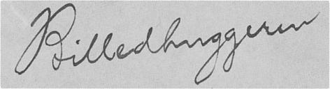Billedhuggeren
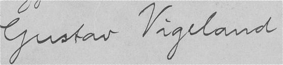Gustav Vigeland
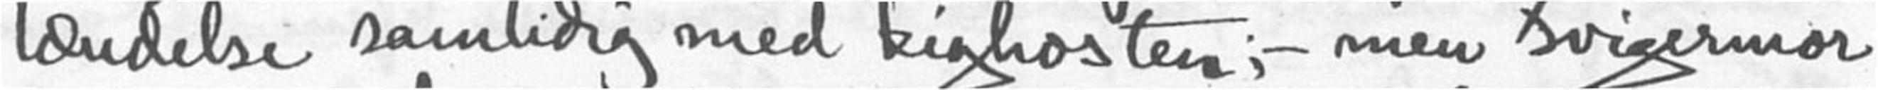tændelse samtidig med kighosten, - men svigermor
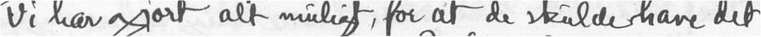Vi har gjort alt muligt for at de skulde have det
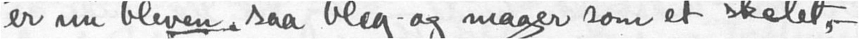er nu bleven saa bleg og mager som et skelet, -
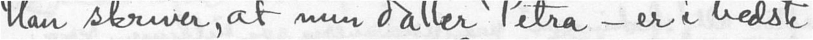Han skriver at min datter Petra - er i bedste
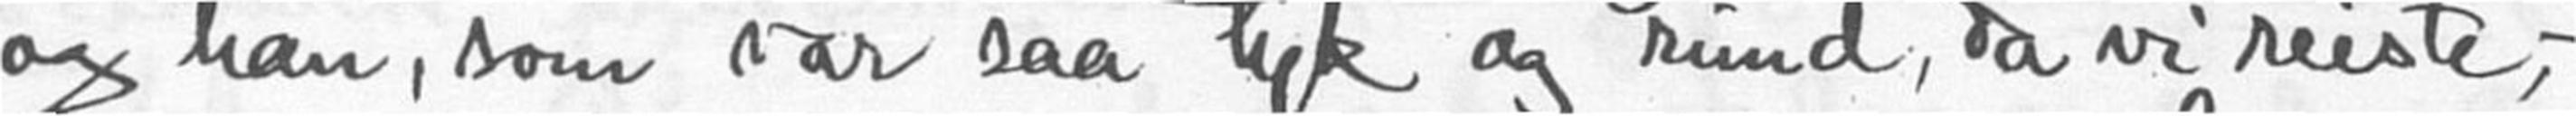og han, som var saa tyk og rund, da vi reiste
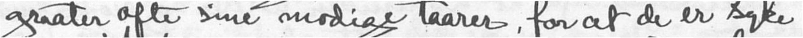graater ofte sine modige taarer, for at de er syke
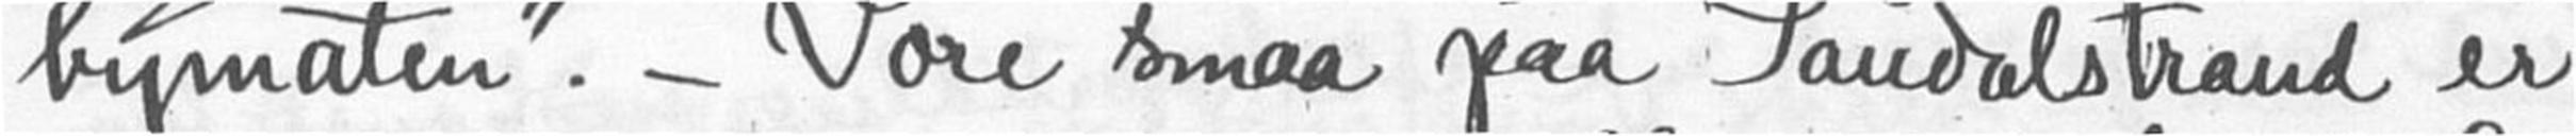bymaten". - Vore smaa paa Sandalstrand er
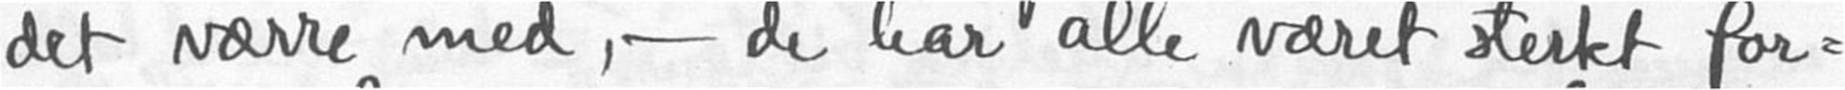det værre med, - de har alle været sterkt for-
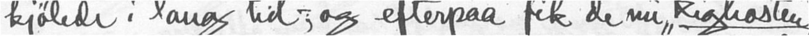kjølede i lang tid ; og efterpaa fik de nu "kighosten"
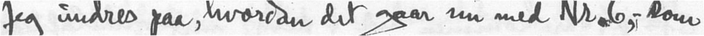Jeg undres paa, hvordan det gaar nu med Nr. 6, -som
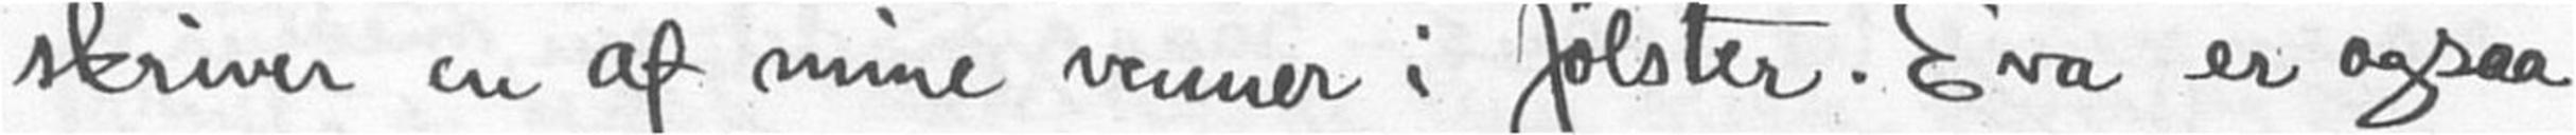skriver en af min venner i Jølster. Eva er ogsaa
